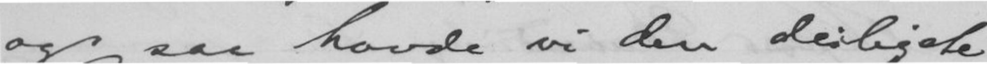og saa havde vi den deiligste
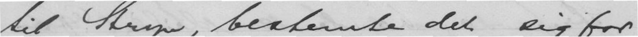til Stryn, bestemte det sig for
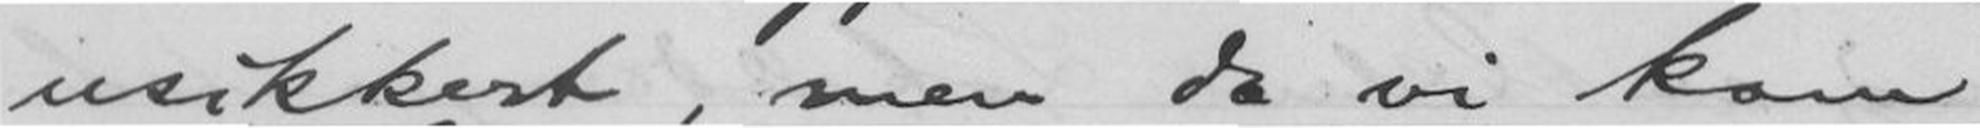usikkert, men da vi kom
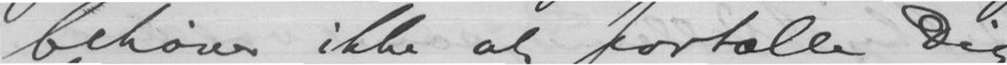behøver ikke at fortælle Dig,
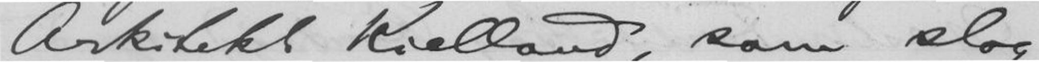Arkitekt Kielland, som slog
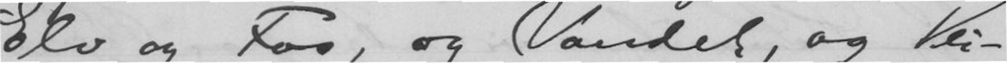Elv og Fos, og Vandet, og Vei-
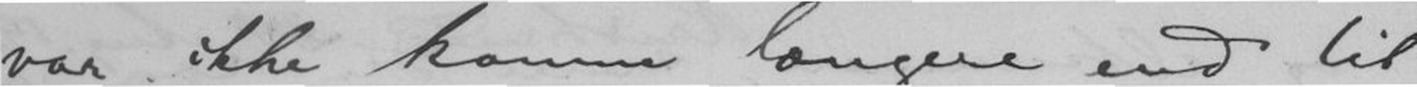var ikke komne lengere end til
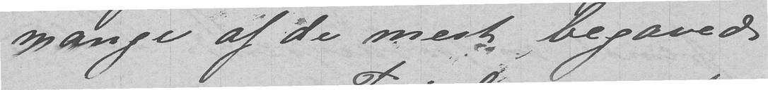mange af de mest begavede
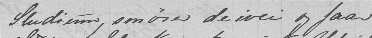Studium, smører de ivei og faar
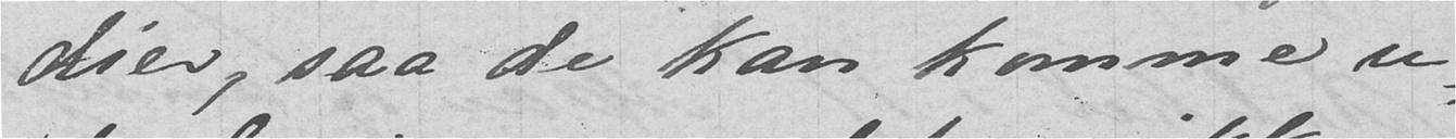dier, saa de kan komme u-
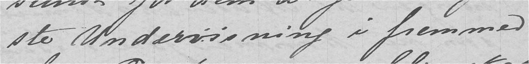ste Undervisning i fremmed
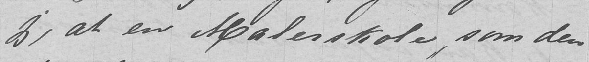jeg, at en Malerskole, som den
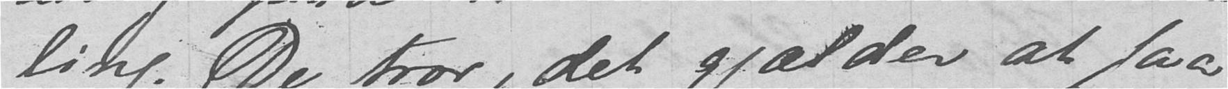ling. De tror, det gjælder at faa
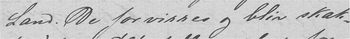Land. De forvirres og blir skak-
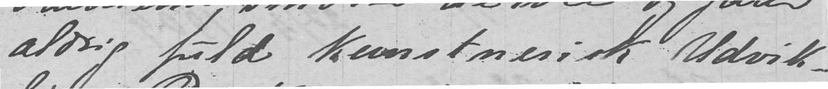aldrig fuld kunstnesisk Udvik-
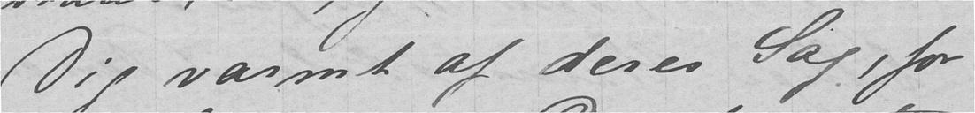Dig varmt af deres Sag, for
